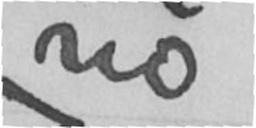no
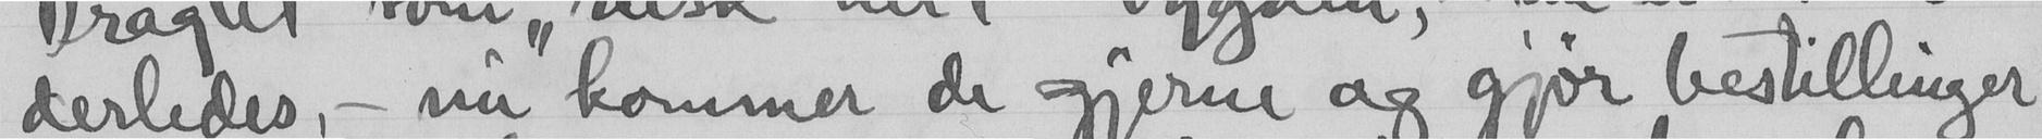derledes, - nu kommer de gjerne og gjør bestillinger
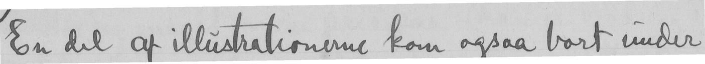En del af illustrationerne kom ogsaa bort under
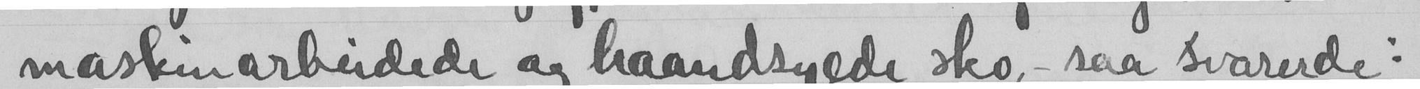maskinarbeidede og haandsyede sko, - saa svarer de:
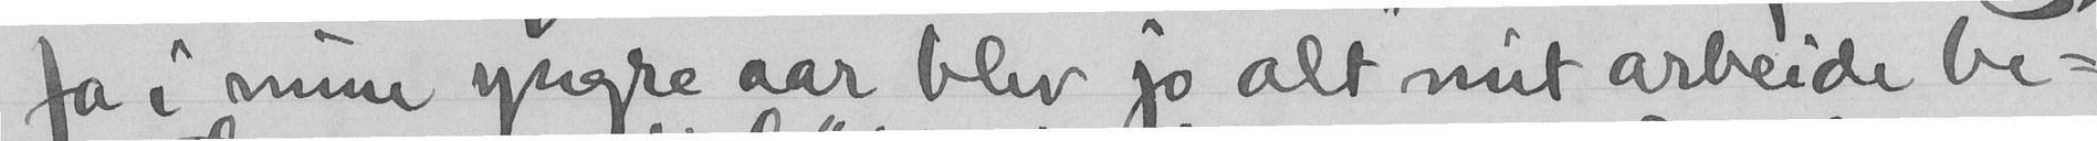Ja i mine yngre aar blev jo alt mit arbeide be-
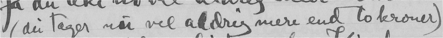(du tager nu vel aldrig mere end to kroner)
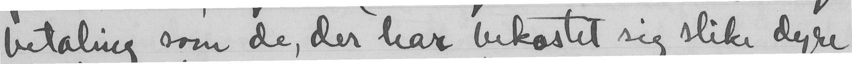betaling som de, der har bekostet sig slike dyre
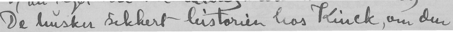De husker sikkert historien hos Kinck, om den
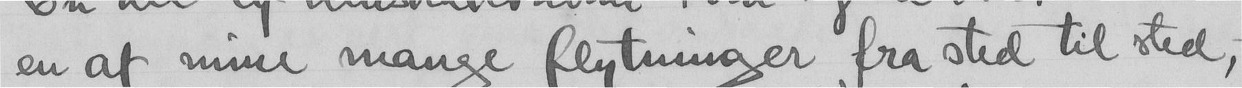en af mine mange flytninger fra sted til sted,-
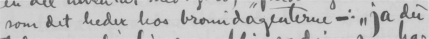som det heder hos bromidagenterne: - "ja du
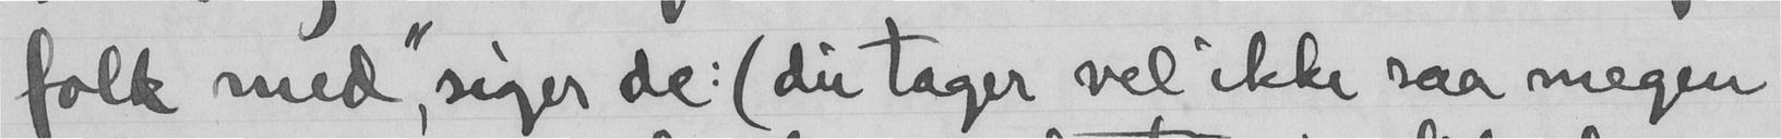folk med", siger de: (du tager vel ikke saa megen
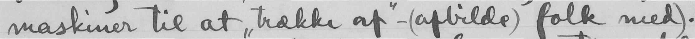maskiner til at "trække af" - (afbilde) folk med).
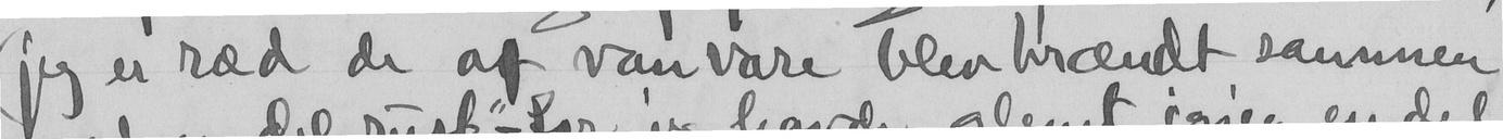(jeg er ræd de af vanvare blev brændt sammen
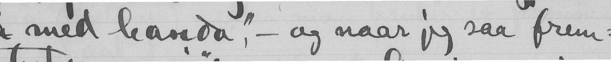med handa, - og naar jeg saa frem-
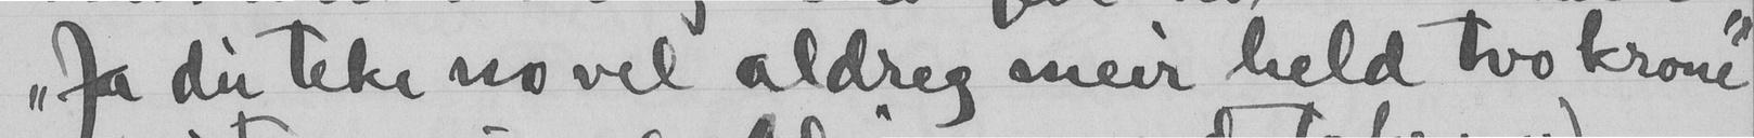"Ja du teke no vel aldrig meir held tvo krone"
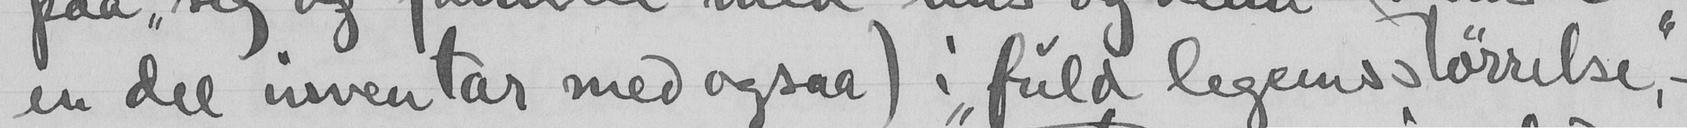en del inventar med ogsaa) i "fuld legemsstørrelse",-
